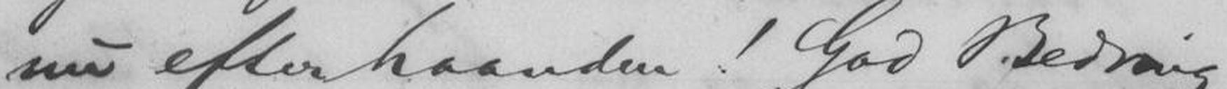nu efterhaanden! God Bedring
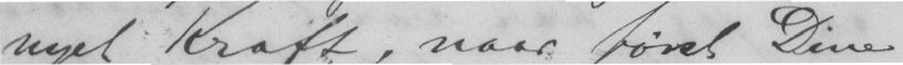nyet Kraft, naar først Dine
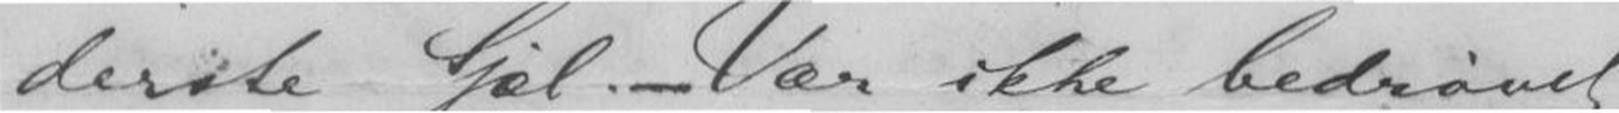derste Sjæl. - Vær ikke bedrøvet,
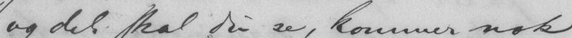og det skal Du se, kommer nok
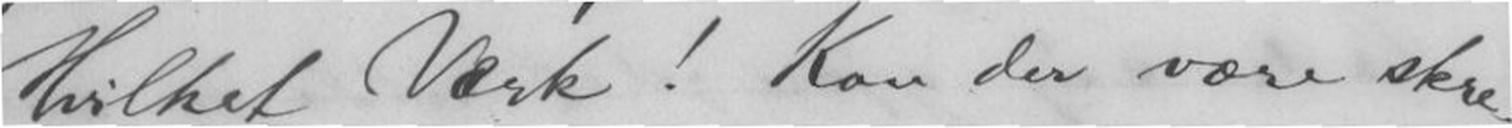Hvilket Værk! Kan der være skre-
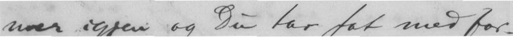mer igjen og Du tar fat med for-
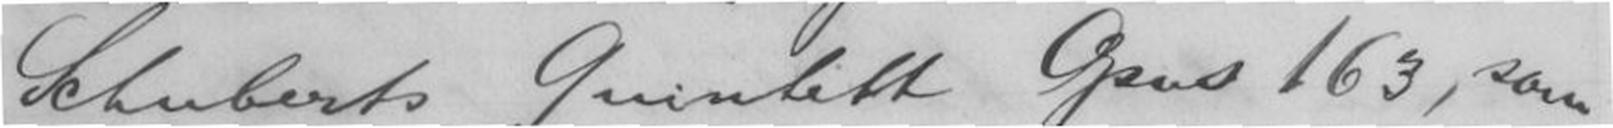Schubert Quintett Opus 163, som
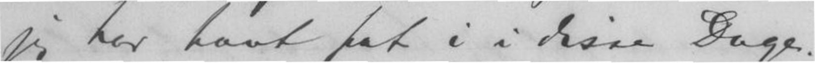jeg har havt fat i i disse Dage.
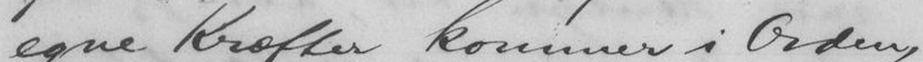egne Kræfter kommer i Orden,
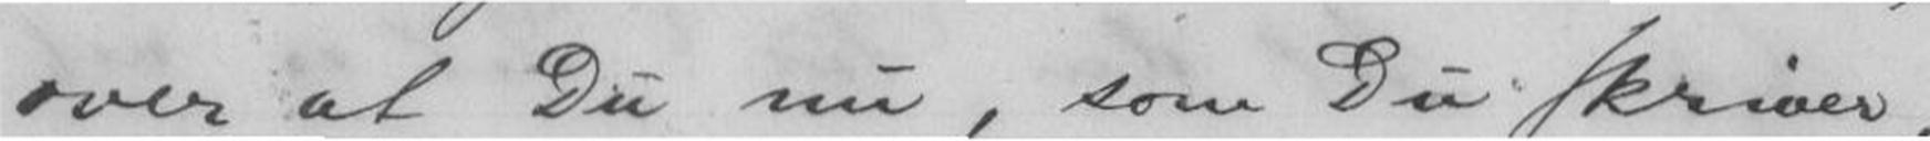over at Du nu, som Du skriver,
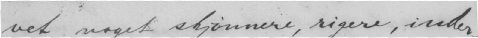vet noget skjønnere, rigere, inder-
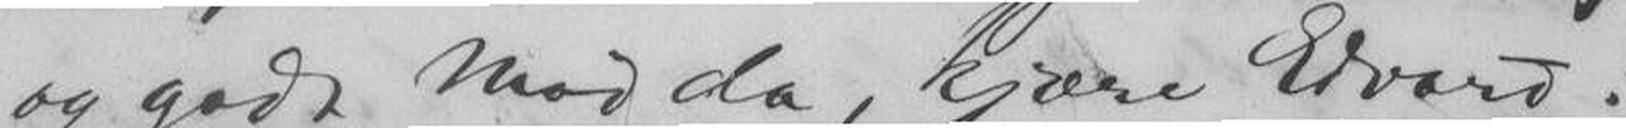og godt Mod da, kjære Edvard!
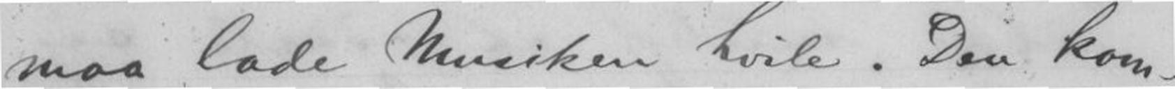maa lade Musiken hvile. Den kom-
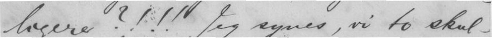ligere?!!! Jeg synes, vi to skul-
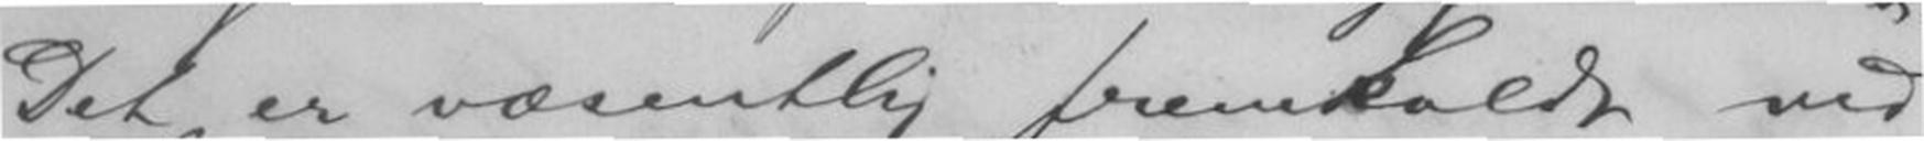Det er væsentlig fremkaldt ved
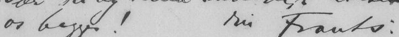os begge! Din Frants.
